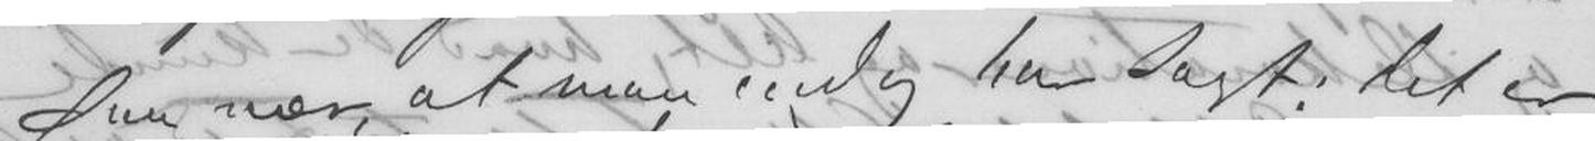saa nær, at man endlig har sagt; det er
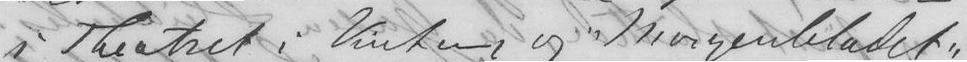i Theatret i Vinter, og Morgenbladet,
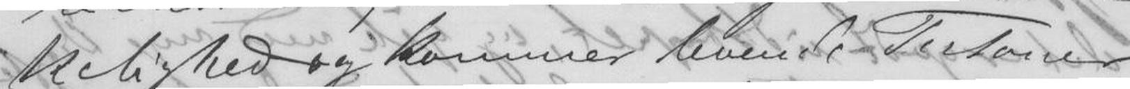kelighed og kommer levende Personer
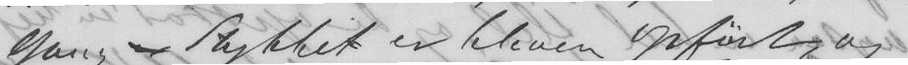Gang Stykket er bleven opført, og
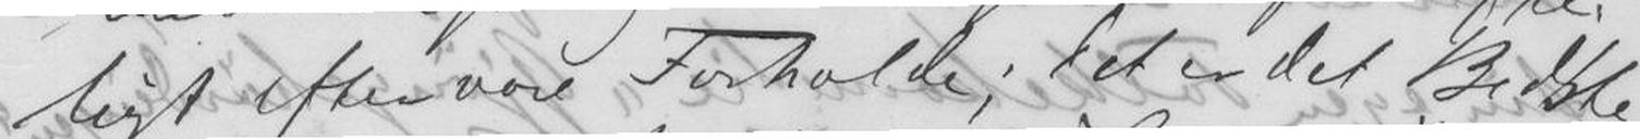ligt efter vore Forholde; det er det Bedste
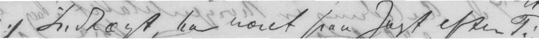til Indtægt, har været paa Jagt efter Pa-
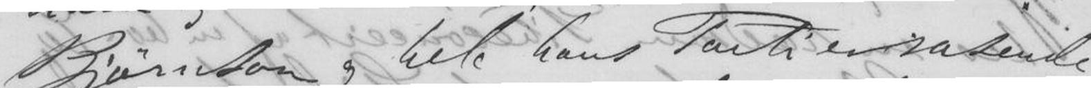Bjørnson og hele hans Parti er rasende
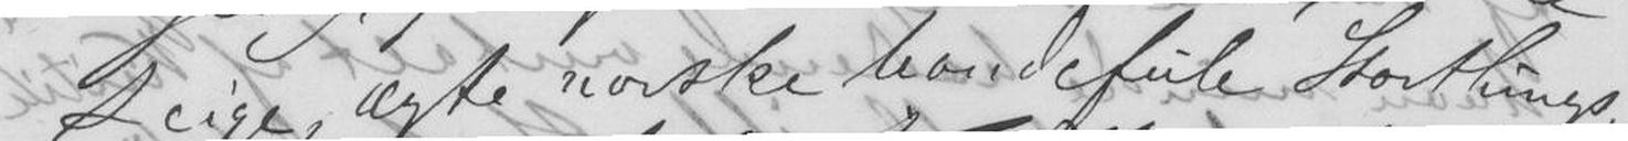seige, ægte norske bondefule Storthings-
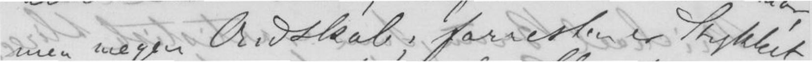men megen Ondskab; forresten er Stykket
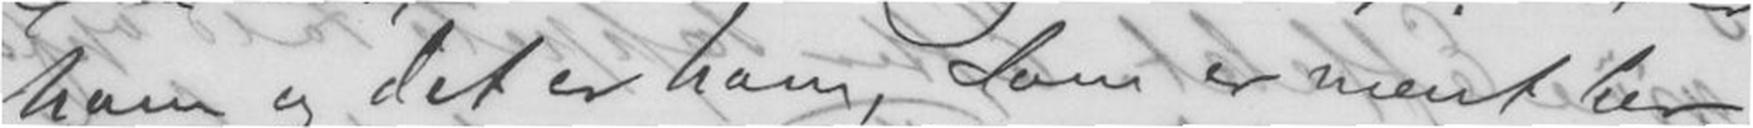ham og det er ham, som er ment her.
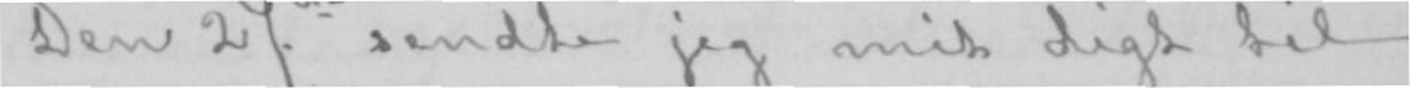Den 27de sendte jeg mit digt til
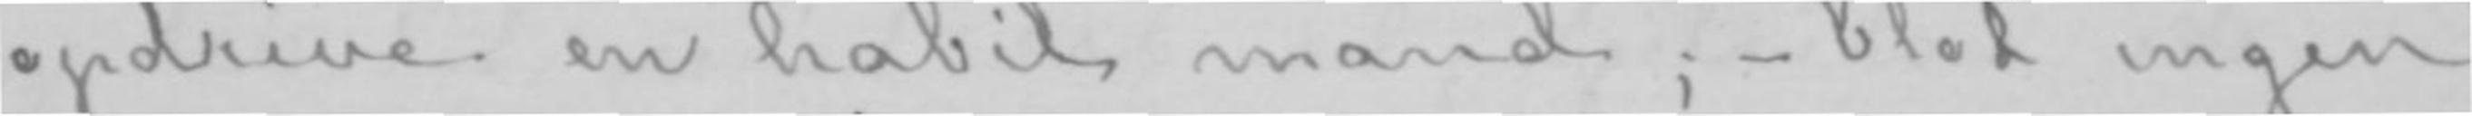opdrive en habil mand;- blot ingen
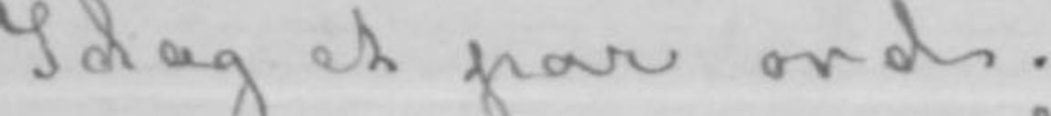Idag et par ord.
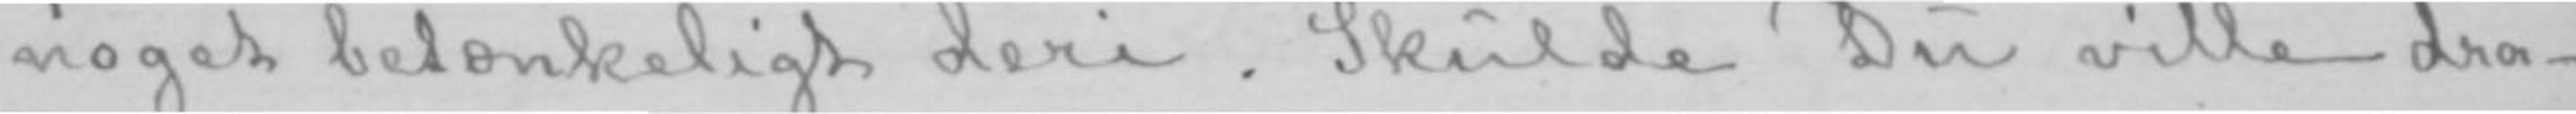noget betænkeligt deri. Skulde Du ville dra-
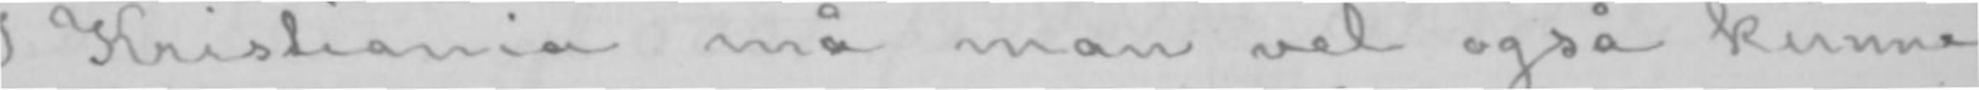I Kristiania må man vel også kunne
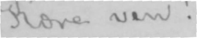Kære ven!
